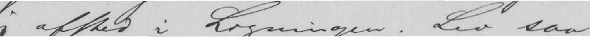jeg afsted i Ligningen. Lev saa
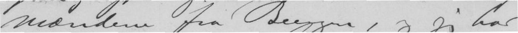mænderne fra Bergen, og jeg har
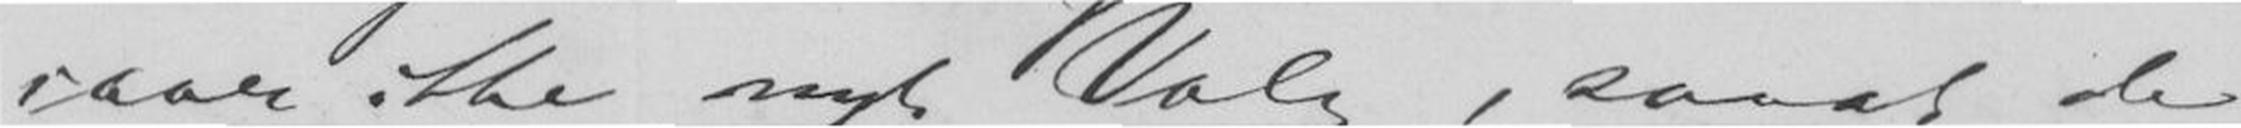iaar ikke nyt Valg, saaat de
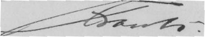Frants.
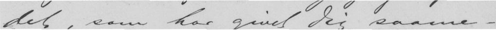det, som har givet dig saame-
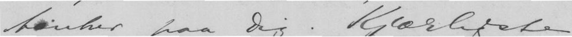tænker paa dig. Kjærligste
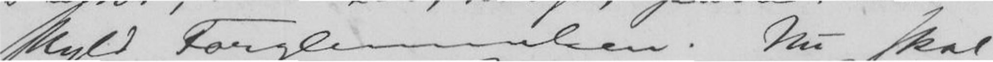skyld Forglemmelsen. Nu skal
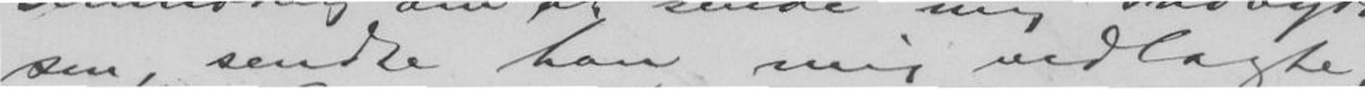sen, sendte han mig vedlagte,
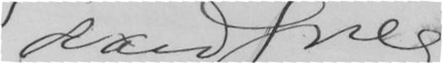Edvard Grieg
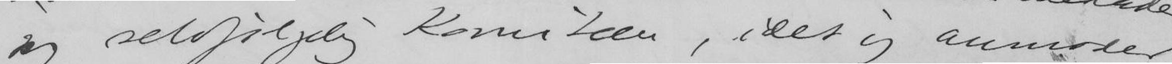jeg selvfølgelig Komiteen, idet jeg anmoder
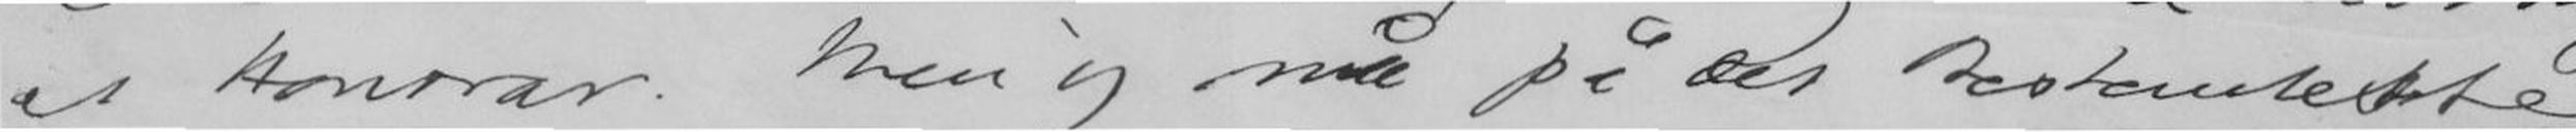et Honorar. Men jeg må på det Bestemteste
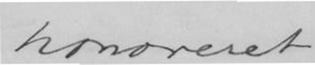er jeg i Samtale med Dem ganske vist gået ud fra
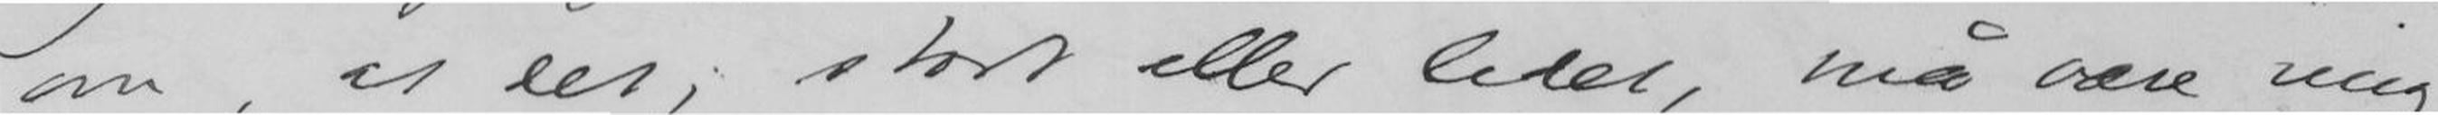om, at det, stort eller lidet, må være mig
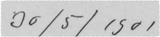30/5/1901
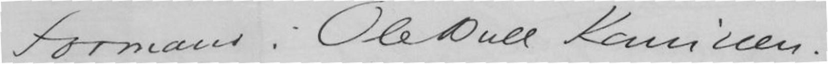Formand i Ole Bull Komiteen.
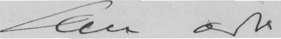Din Ærb
